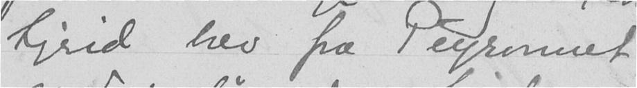Sigrid brev fra Peyronnet
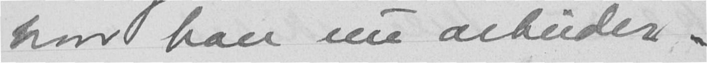hvor han nu arbeider.
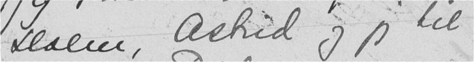Holm, Astrid og jeg til
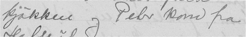kjøkken og Peter kom fra
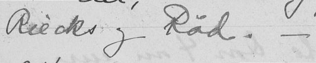Riecks og Rød. -
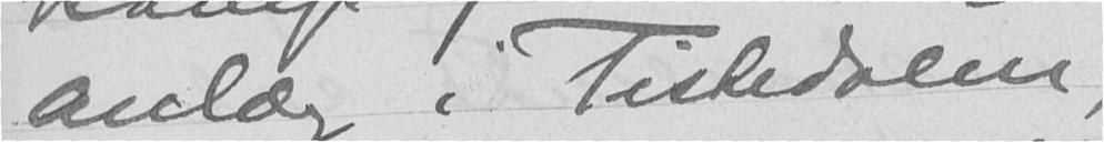anlæg i Tistedalen,
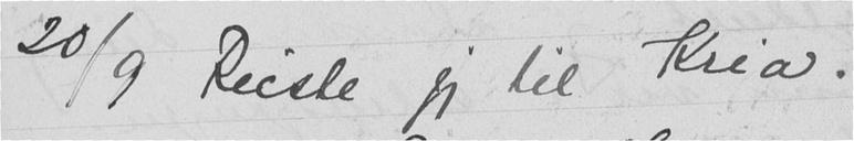20/9 Reiste jeg til Kria.
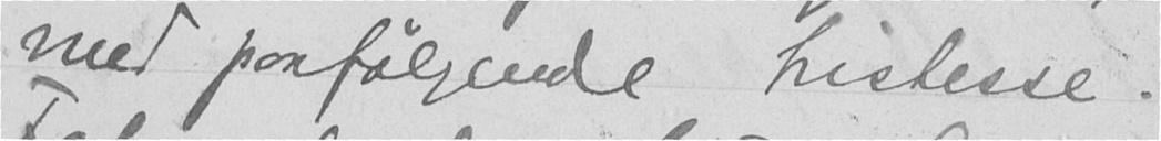med paafølgende tristesse.
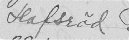Hafsrød.
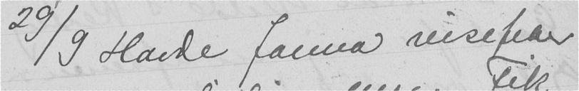29/9 Havde Janna reisefeber
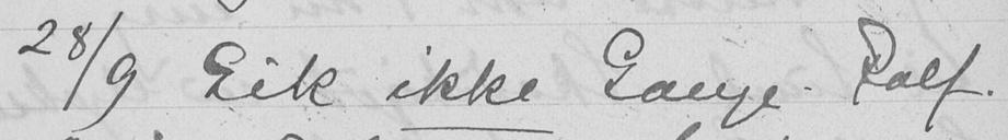28/9 Gik ikke Gange Rolf.
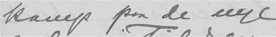kamp paa de nye
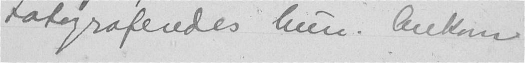Fotograferedes hun. Ankom
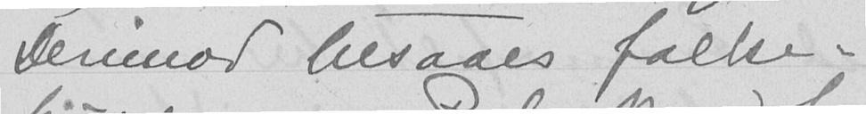Derimod besaaes folke-
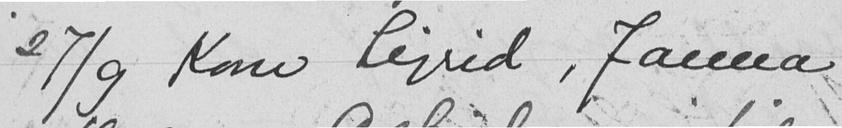27/9 Kom Sigrid, Janna
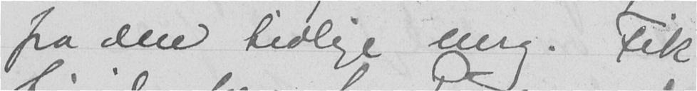fra den tidlige mrg. Fik
